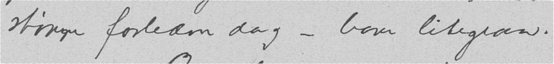større forleden dag - bare litegran.
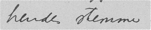hendes stemme
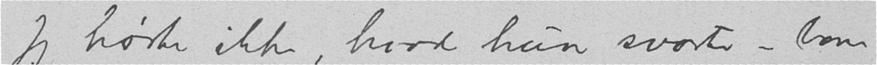Jeg hørte ikke, hvad hun svarte – bare
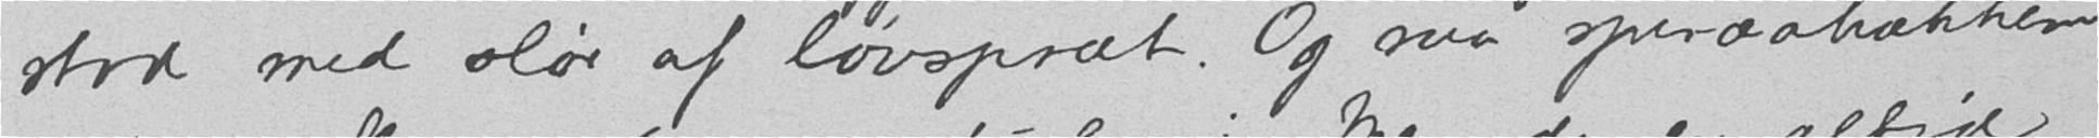stod med slør af løvspræt. Og saa spiræahækken
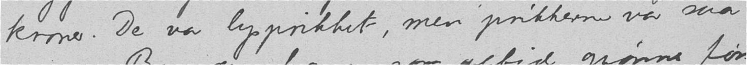kroner. De var lysprikket, men prikkerne var saa
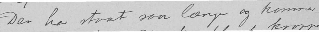Den har staat saa længe og kommer
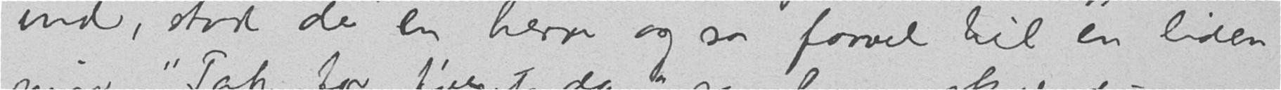ind, stod der en herre og sa farvel til en liden
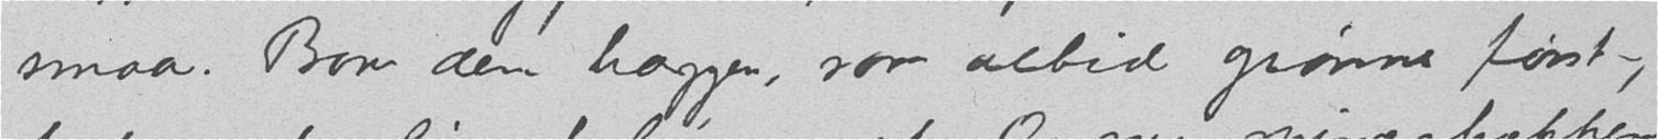smaa. Bare den hæggen, sov altid grønne først -,
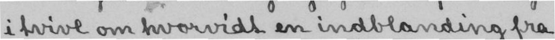i tvivl om hvorvidt en indblanding fra
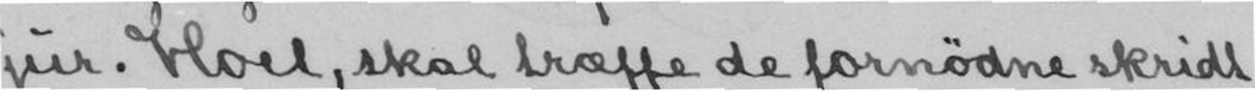jur. Hoel, skal træffe de fornødne skridt
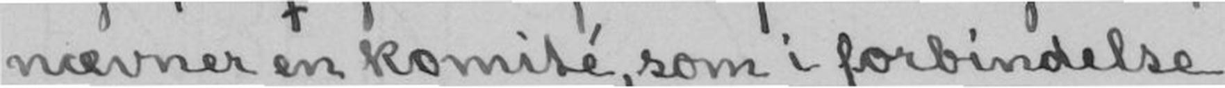nævner en komité, som i forbindelse
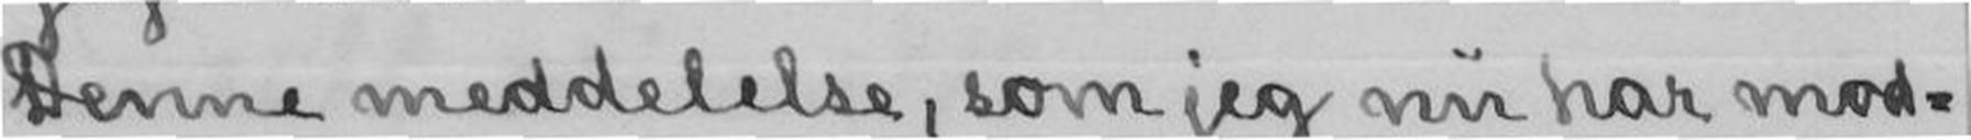Denne meddelelse, som jeg nu har mod-
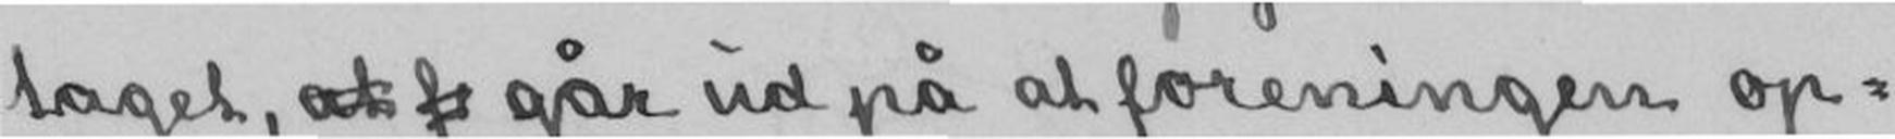taget, at f går ud på at foreningen op-
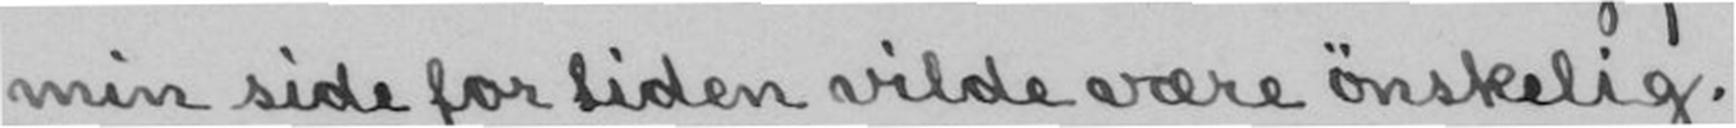min side for tiden vilde være ønskelig.
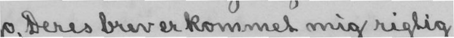Jo, Deres brev er kommet mig rigtig
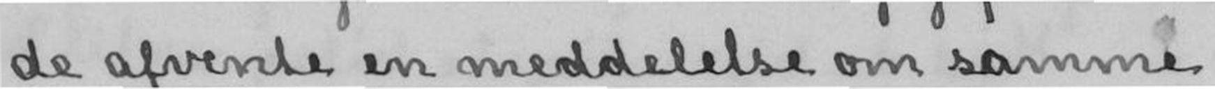de afvente en meddelelse om samme
